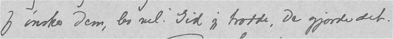Jeg ønsker Dem, lev vel. Gid jeg trodde, De gjorde det.
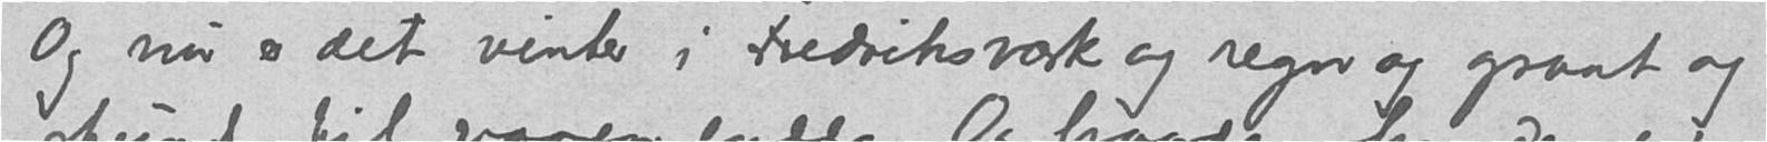- Og nu er det vinter i Fredriksværk og regn og graat og
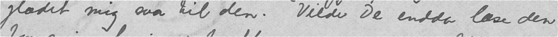glædet mig saa til den. Vilde De endda læse den
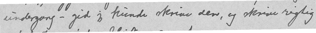undergang - gid jeg kunde skrive den, og skrive rigtig
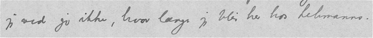jeg ved jo ikke, hvor længe jeg blir her hos Lehmanns.
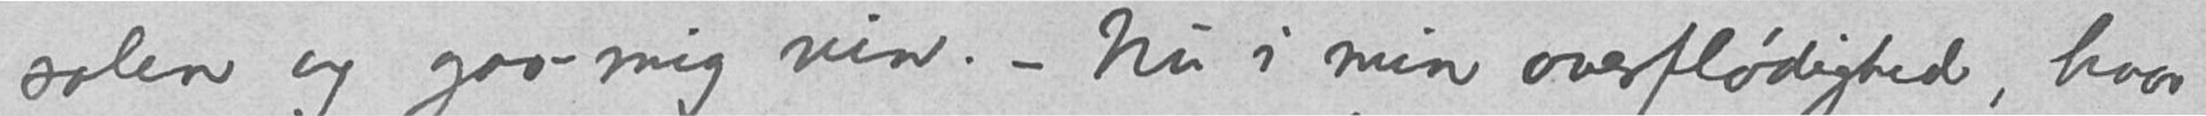solen og gav mig vin. - Nu i min overflødighed, hvor
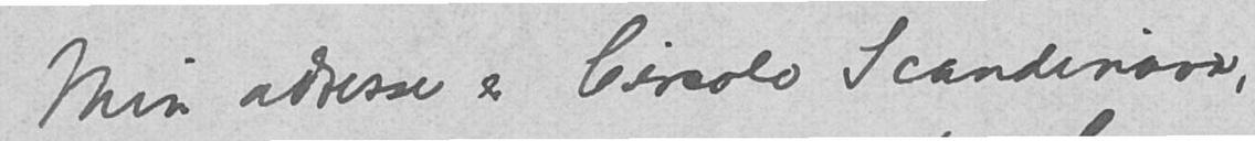Min adresse ir Circolo Scandinavo,
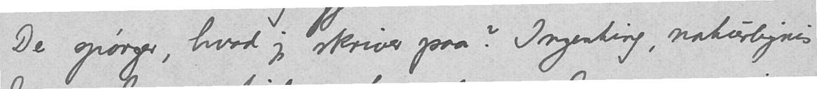De spørger, hvad jeg skriver paa? Ingenting, naturligvis
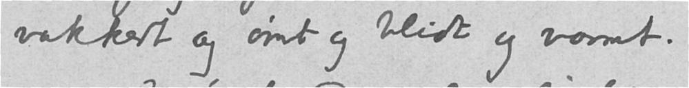vakkert og ømt og blidt og varmt.
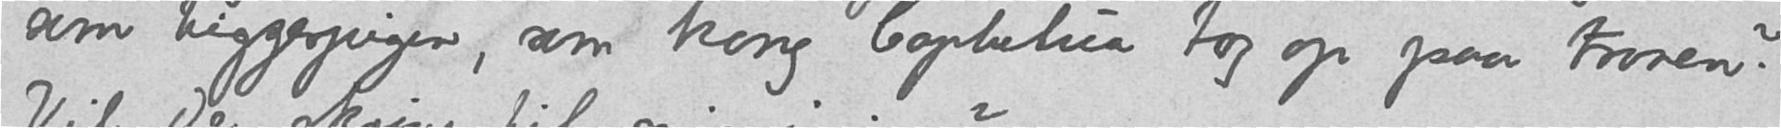som tiggerpigen, som kong Cophetua tog op paa tronen?
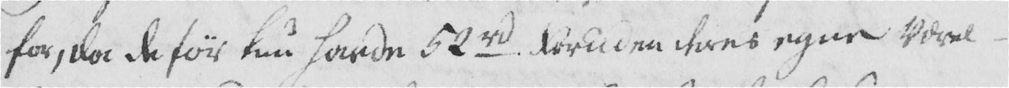for, da de før kun havde 52 rd. Foruden deres egne Værel-
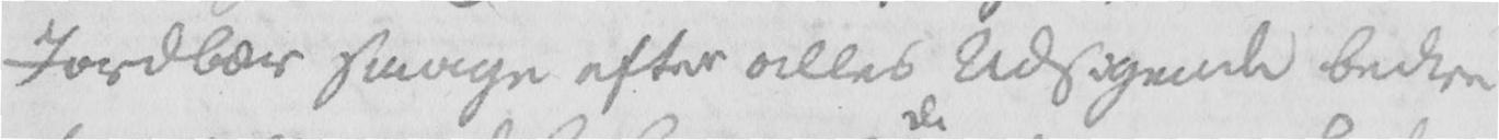Jordbær smage efter alles Udsigende bedre
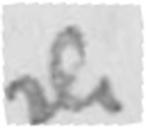de
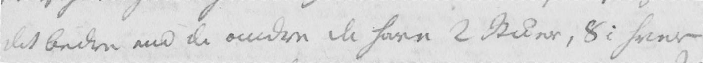det bedre end de andre de have 2 Stuer, 8 i hver
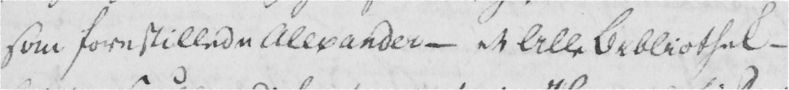som forestillede Alexander - et lille Bibliothek -
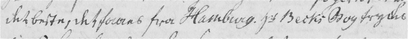det beste, det faaes fra Hamburg. Hr Becks Bog tryckes
